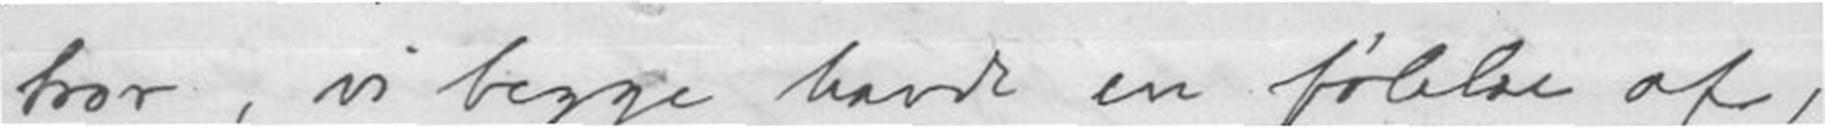tror, vi begge havde en følelse af,
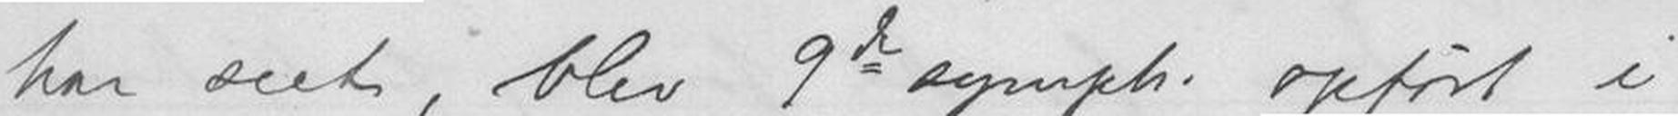har seet, blev 9de symfph. opført i
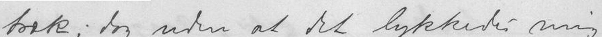trek; dog uden at det lykkedes mig
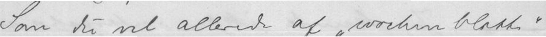Som du vel allerede af "Wochen blatt"
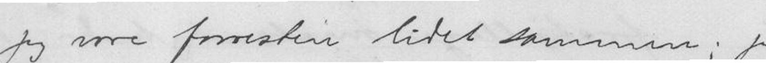jeg vare forresten lidet sammen; jeg
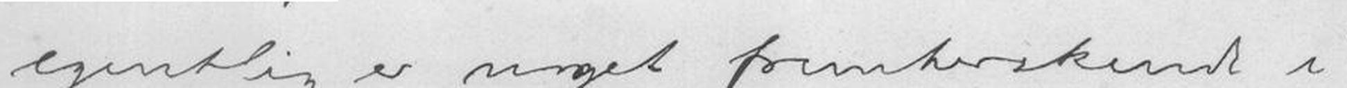egentlig er noget fremherskende i
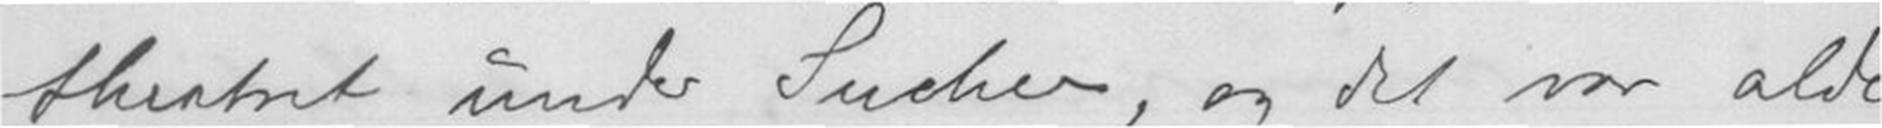theatret under Sucher, og det var alde
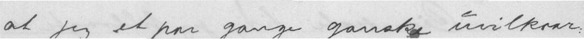at jeg et par gange ganske uvilkaar
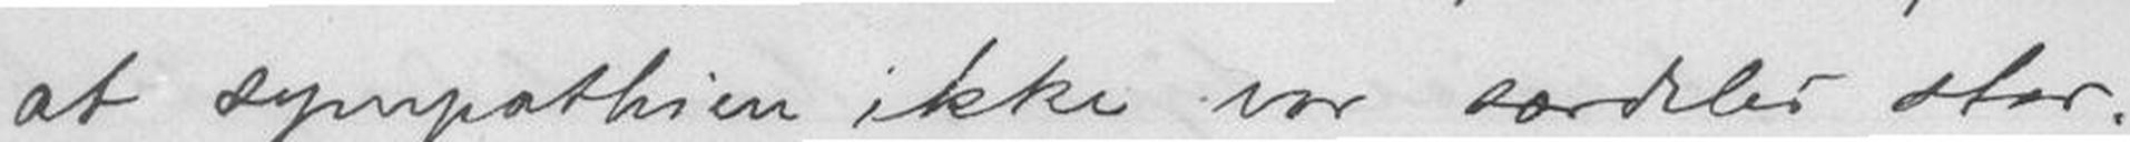at sympathien ikke var særdeles stor.
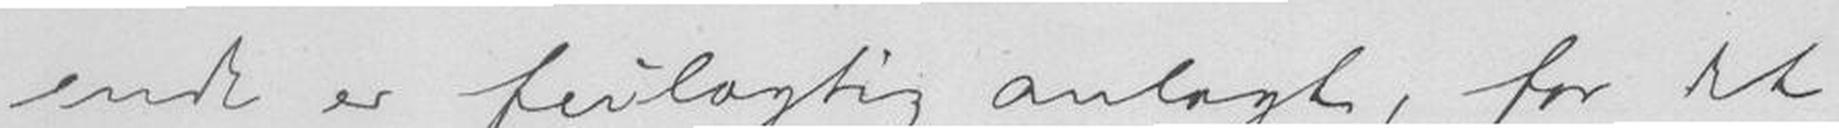ende er feilagtig anlagt, for det
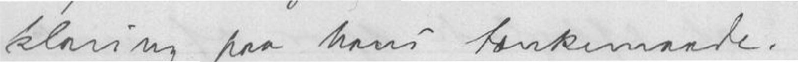klaring paa hans tænkemaade.
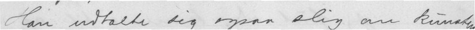Han udtalte sig ogsaa slig om kunsten,
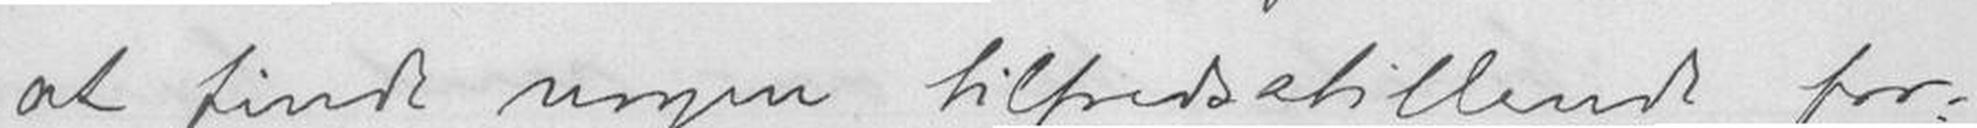at finde nogen tilfredestillende for-
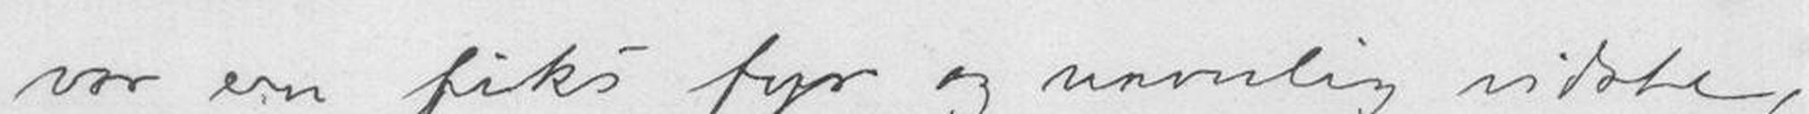var ene fiks fyr og navnlig vidste,
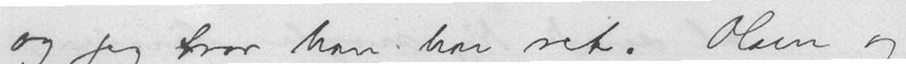og jeg tror, han har ret. Ham og
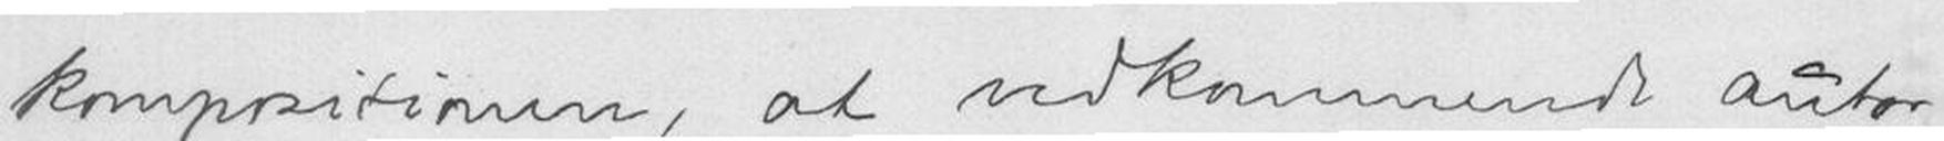kompositionen, at vedkommende autor
